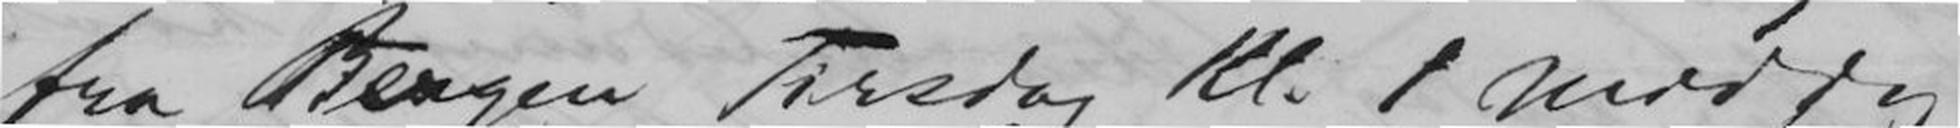fra Bergen Tirsdag Kl. 1 Middag
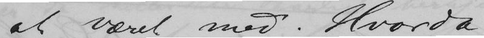at været med. Hvorda!
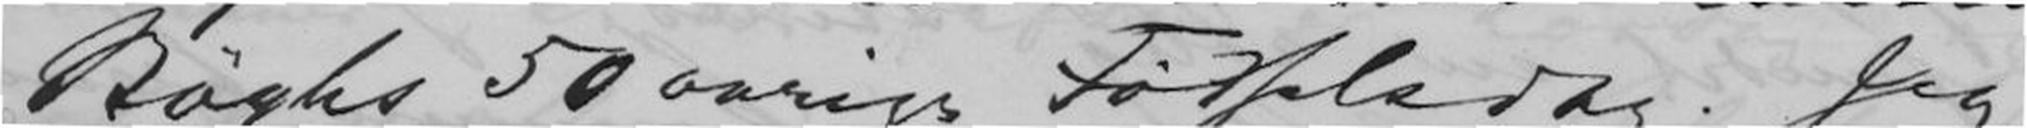Bøghs 50 aarige Fødselsdag. Jeg
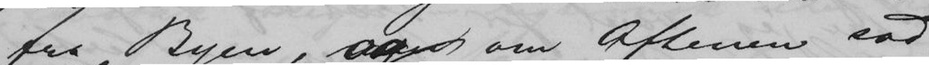fra Byen, og om Aftenen sad
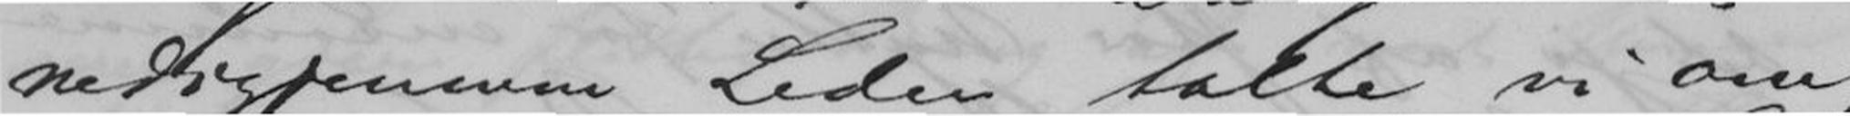nedigjennem Leden talte vi om,
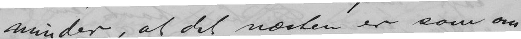minder, at det næsten er som om
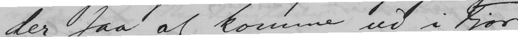der saa at komme ud i Fjor-
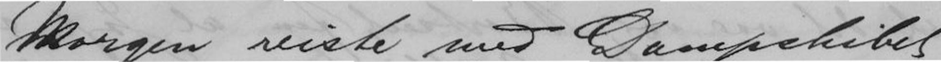Morgen reiste med Dampskibet
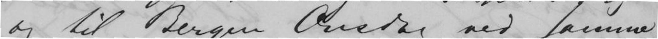og til Bergen Onsdag ved samme
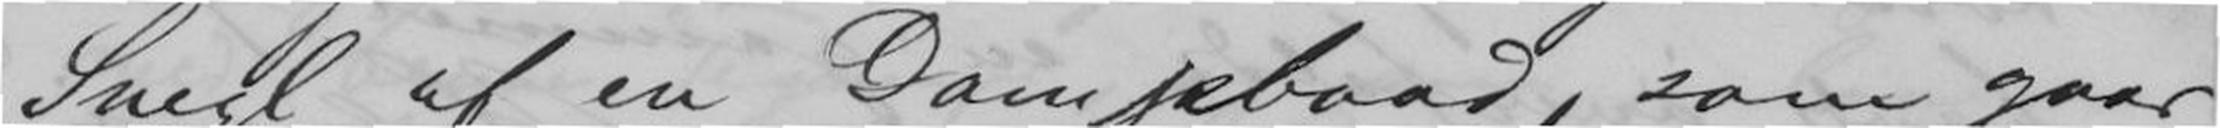Snegl af en Dampebaad, som gaar
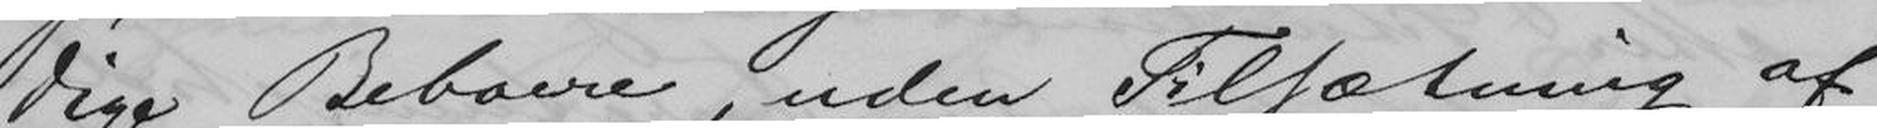dige Beboere, uden Tilsætning af
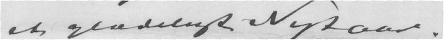et glædeligt Nytaar!
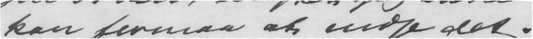kan formaa at indsa det.
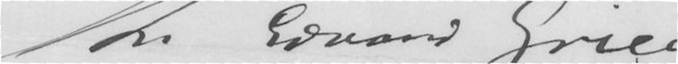Hr Edvard Grieg.
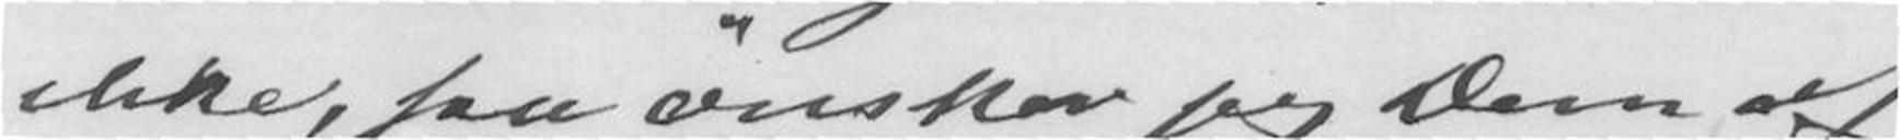ikke, saa ønsker jeg Dem af
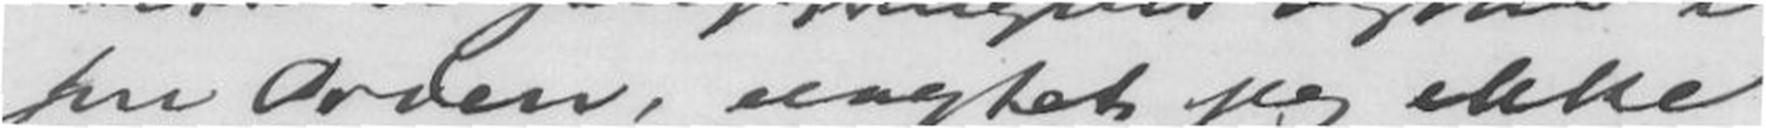sin Orden, uagtet jeg ikke
